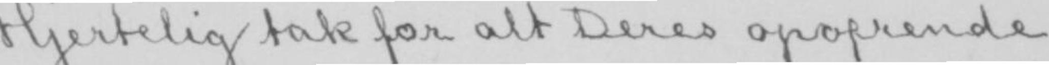Hjertelig tak for alt Deres opofrende
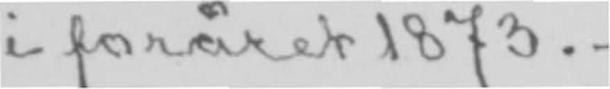i foråret 1873.-
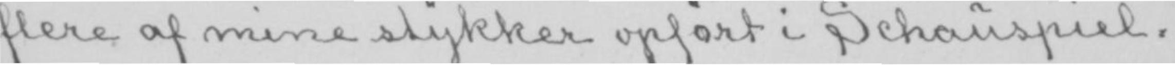flere af mine stykker opført i Schauspiel-
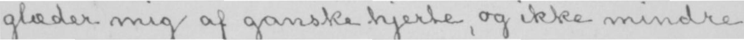glæder mig af ganske hjerte, og ikke mindre
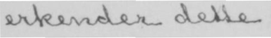erkender dette.
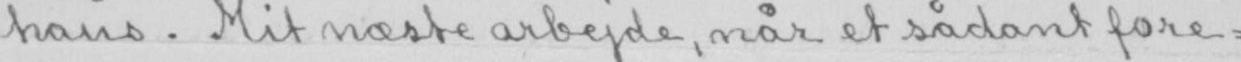haus. Mit næste arbejde, når et sådant fore-
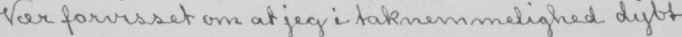Vær forvisset om at jeg i taknemmelighed dybt
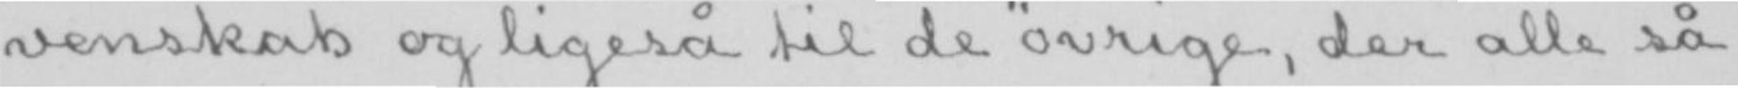venskab og ligeså til de øvrige, der alle så
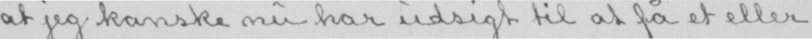at jeg kanske nu har udsigt til at få et eller
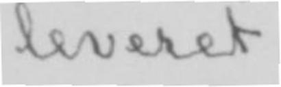leveret.
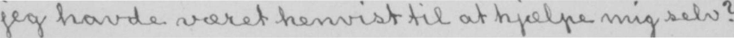jeg havde været henvist til at hjælpe mig selv?
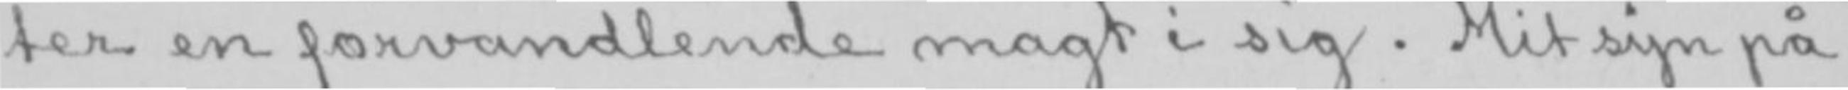ter en forvandlende magt i sig. Mit syn på
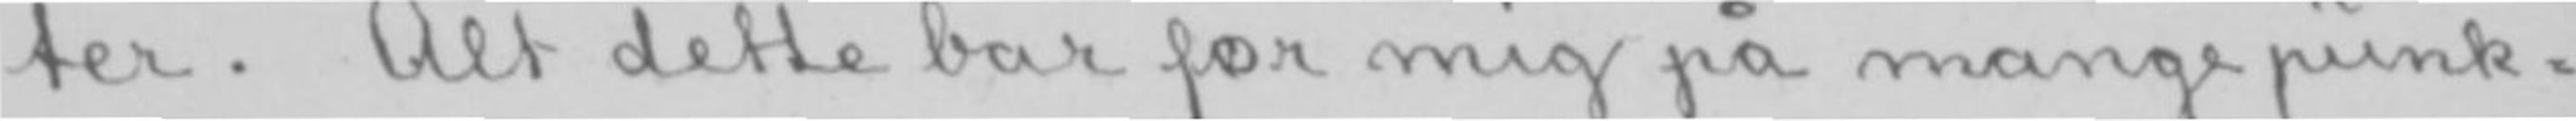ter. Alt dette bar for mig på mange punk-
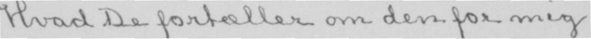Hvad De fortæller om den for mig
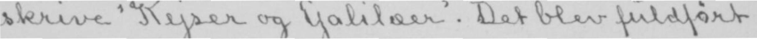skrive «Kejser og Galilæer». Det blev fuldført
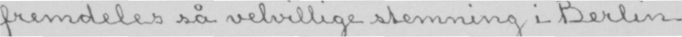fremdeles så velvillige stemning i Berlin
